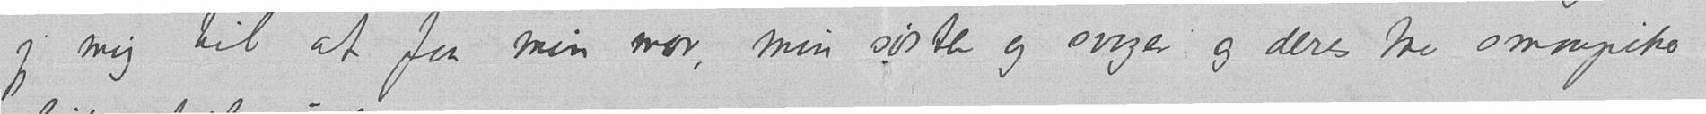jeg mig til at faa min mor, min søster og svoger og deres tre smaapiker
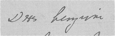Deres hengivne
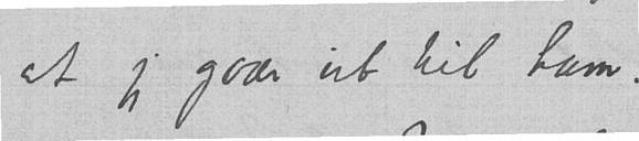at jeg gaar ut til ham.
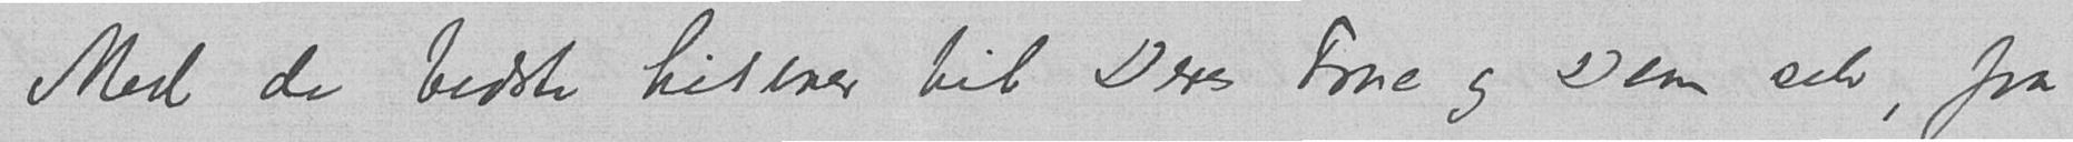Med de bedste hilsener til Deres Frue og Dem selv, fra
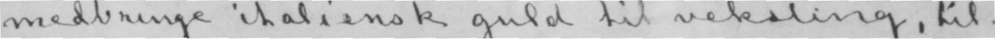medbringe italiensk guld til veksling, til-
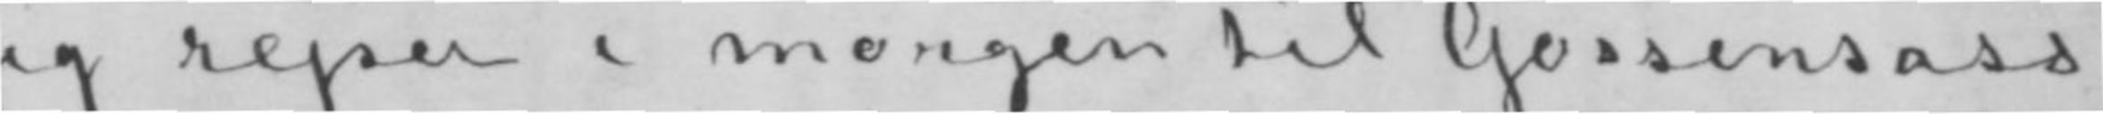Jeg rejser i morgen til Gossensass
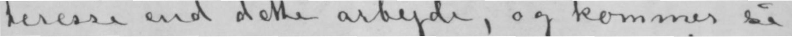teresse end dette arbejde, og kommer så-
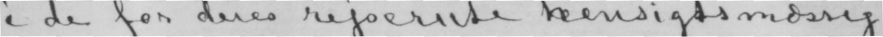i de for deres rejserute hensigtsmæssig-
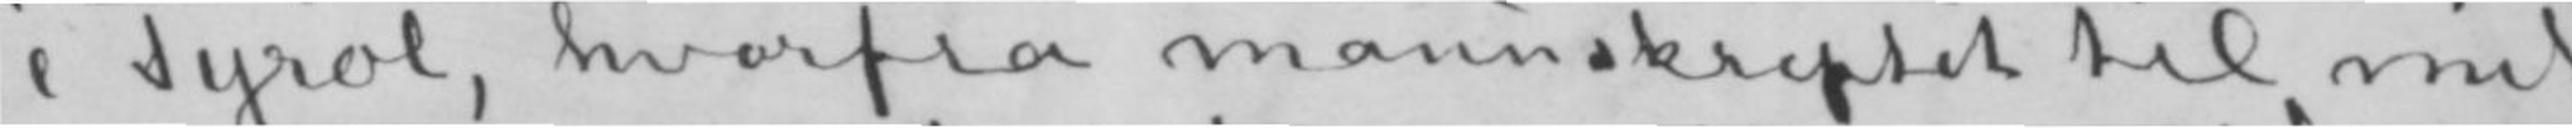i Tyrol, hvorfra manuskriptet til mit
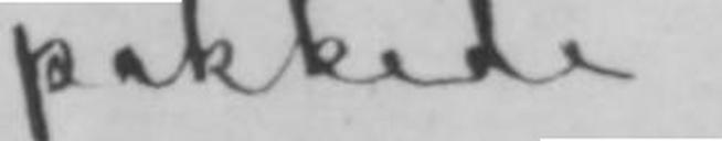pakkede.
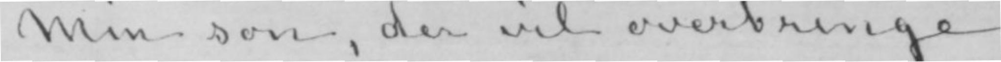Min søn, der vil overbringe
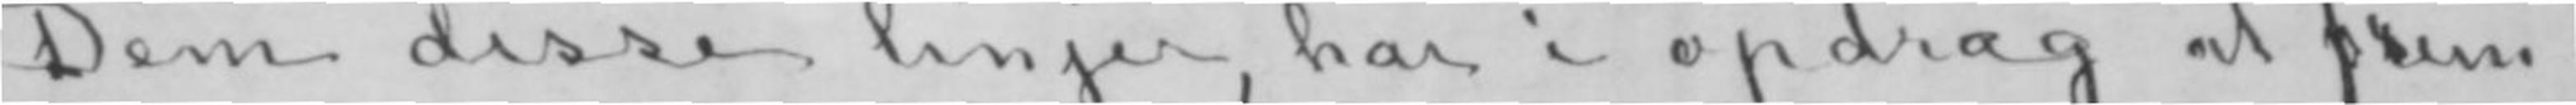Dem disse linjer, har i opdrag at frem-
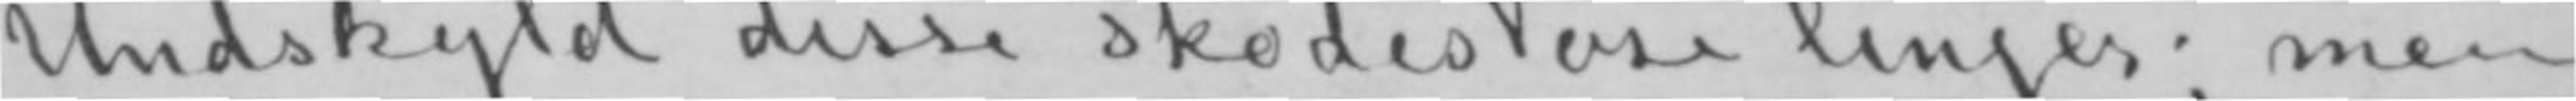Undskyld disse skødesløse linjer; men
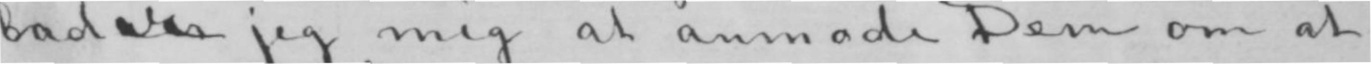lader jeg mig at anmode Dem om at
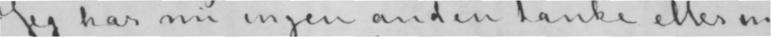Jeg har nu ingen anden tanke eller in-
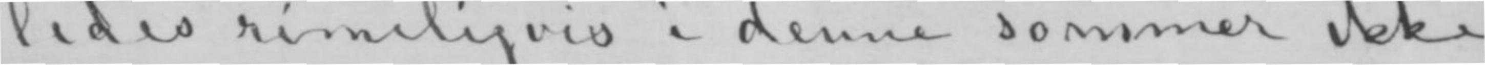ledes rimeligvis i denne sommer ikke
